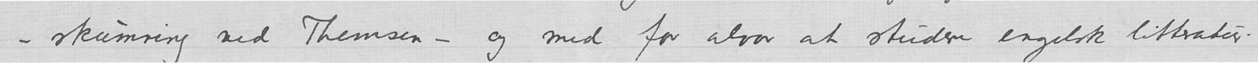- skumring ved Themsen - og med for alvor at studere engelsk litteratur.
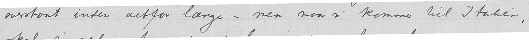overstaat inden altfor længe - men naar vi kommer til Italien,
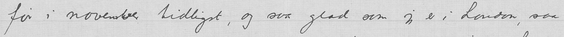før i november tidligst, og saa glad som jeg er i London, saa
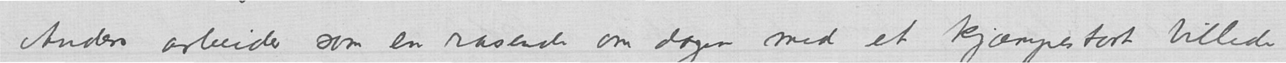Anders arbeider som en rasende om dagen med et kjæmpestort billede
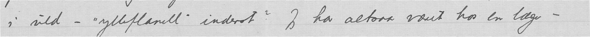i uld - "ylleflanell" inderst? Jeg har altsaa været hos en læge -
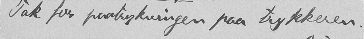Tak for paatrykningen paa trykkeren.
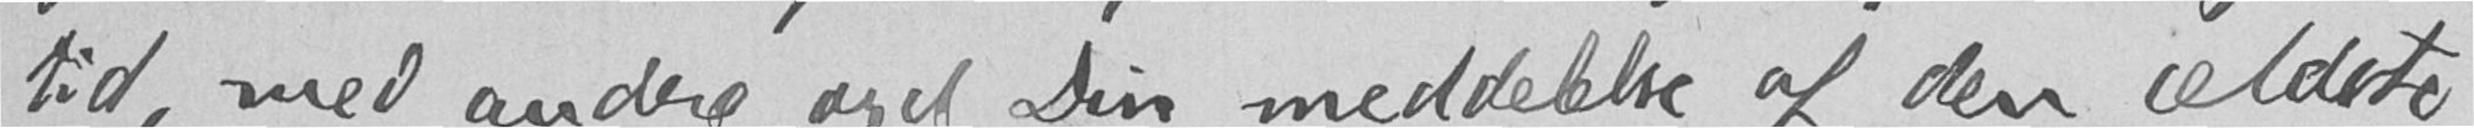tid, med andre ord Din meddelelse af den ældste
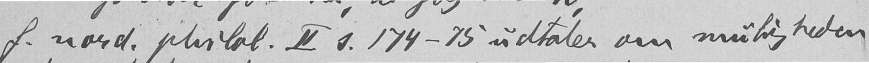f. nord. philol. II s. 174-75 udtaler om muligheden
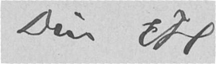Din EH.
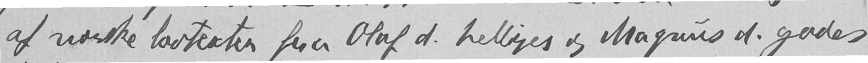af norske lovtexter fra Olaf d. helliges og Magnus d. godes
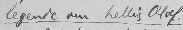legende om hellig Olaf.
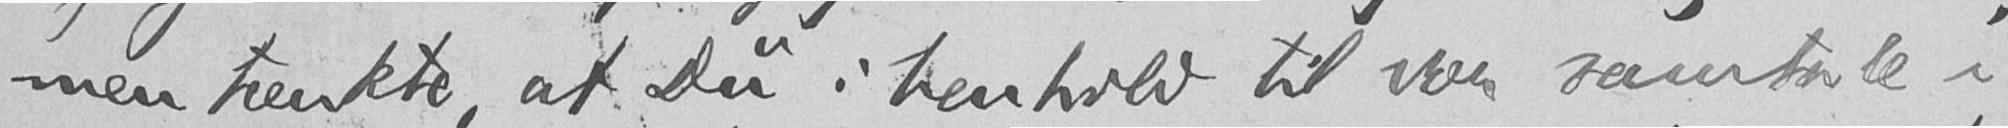men tænkte, at Du i henhold til vor samtale i
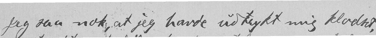Jeg saa nok, at jeg havde udtrykt mig klodset,
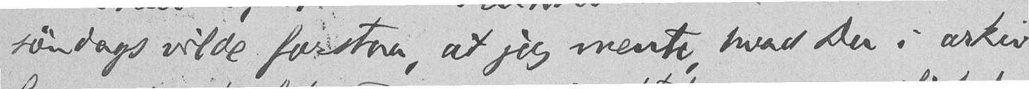søndags vilde forstaa, at jeg mente, hvad Du i arkiv
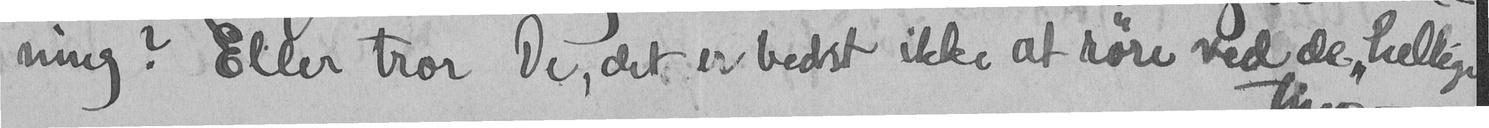ning? Eller tror De, det er bedst ikke at røre ved de "hellige
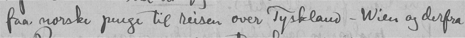faa norske penge til reisen over Tyskland - Wien og derfra
